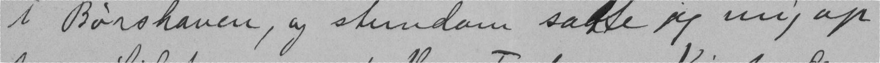i Børshaven, og stundom satte jeg mig op
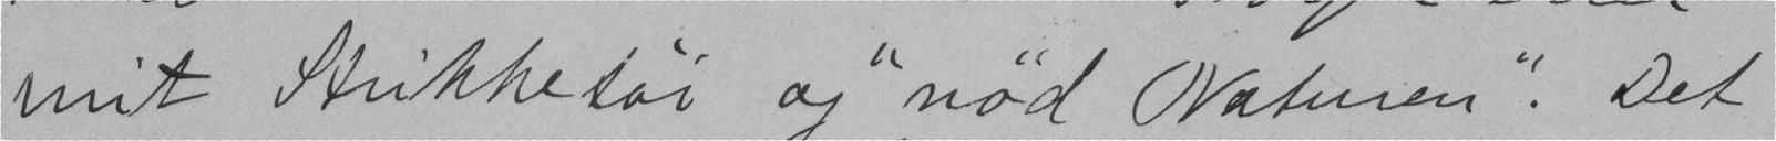mit Strikketøi og "nød Naturen". Det
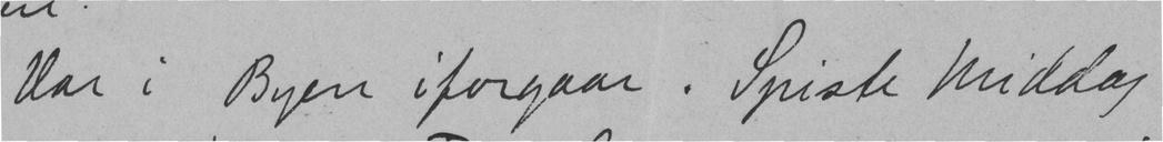Var i Byen iforgaar. Spiste Middag
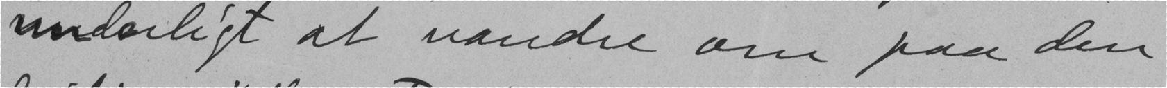underligt at vandre om paa den
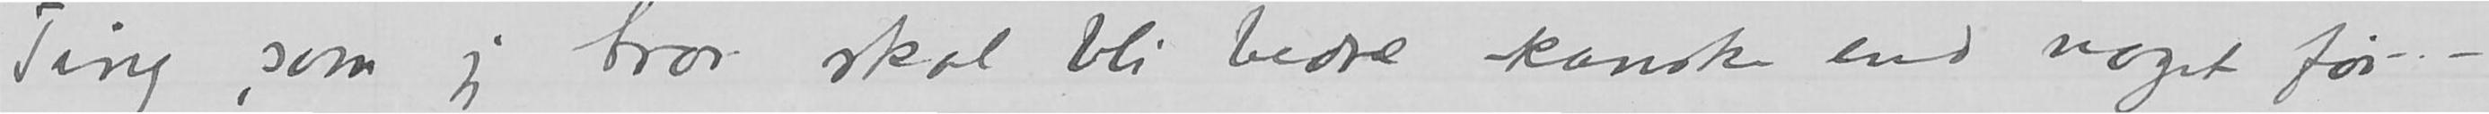Ting, som jeg tror skal bli bedre kanske end noget før –.
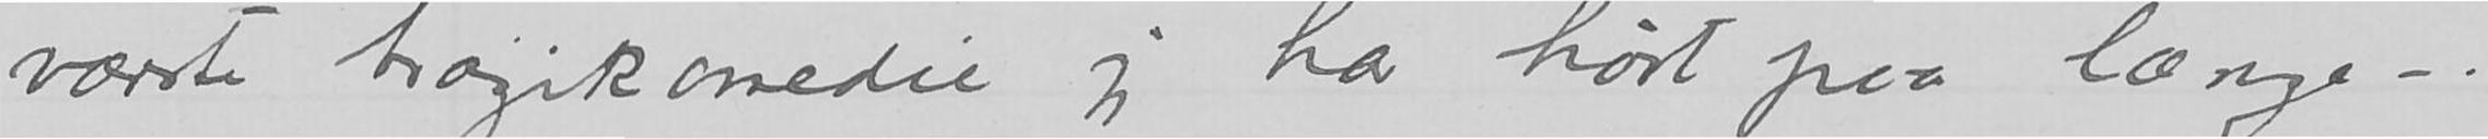værste tragikomedie jeg har hørt paa længe –.
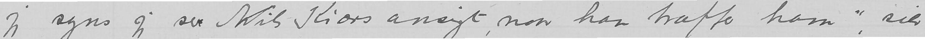«jeg synes jeg ser Nils Kiærs ansigt, naar han træffer ham», sier
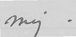mig.
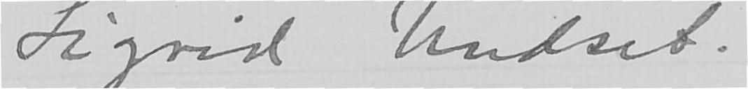Sigrid Undset.
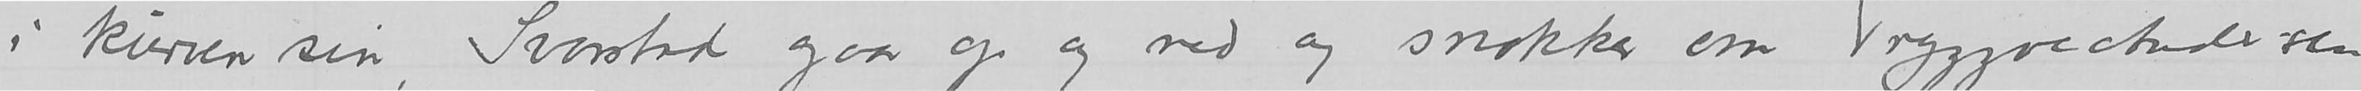i kurven sin, Svarstad gaar op og ned og snakker om Trygve Andersen
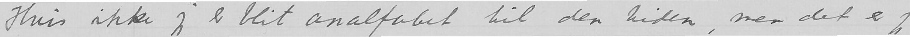Hvis ikke jeg er blit analfabet til den tiden, men det er jeg
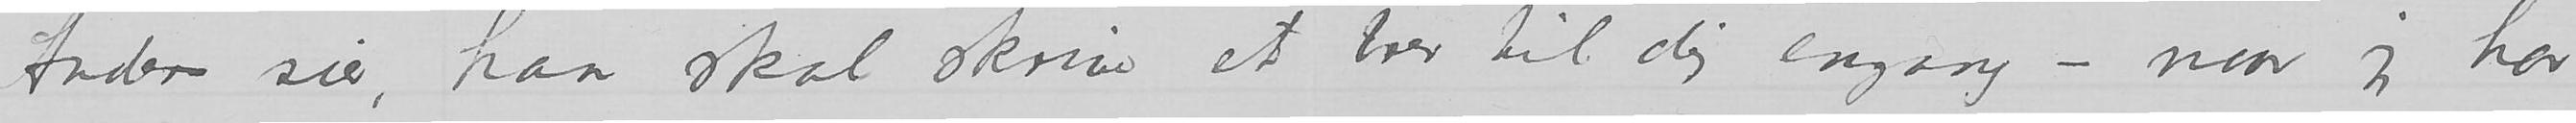Anders sier, han skal skrive et brev til dig engang – naar jeg har
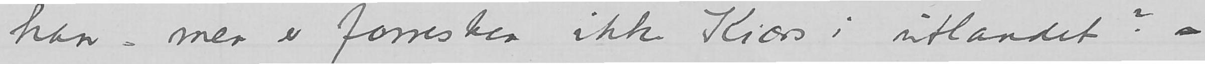han – men er forresten ikke Kiærs i utlandet? –
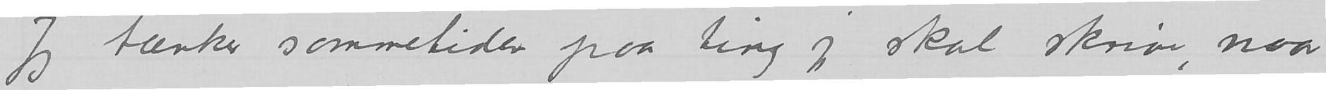Jeg tænker sommetider paa ting jeg skal skrive, naar
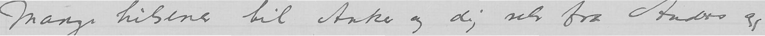Mange hilsener til Anker og dig selv fra Anders og
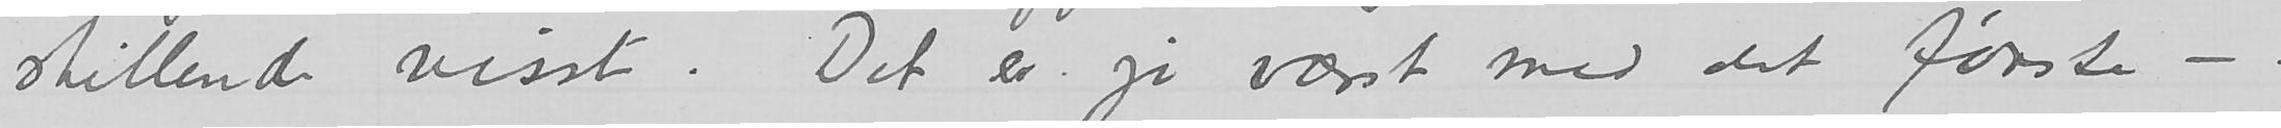stillende visst. Det er jo verst med det første –.
